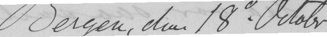Bergen, den 18d Octobr
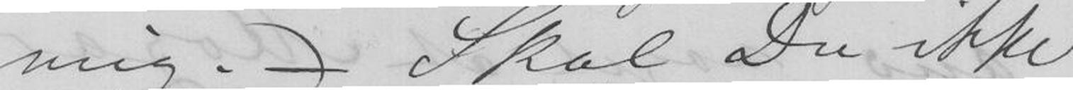mig.) - Skal Du ikke
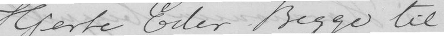Hjerte Eder Begge til
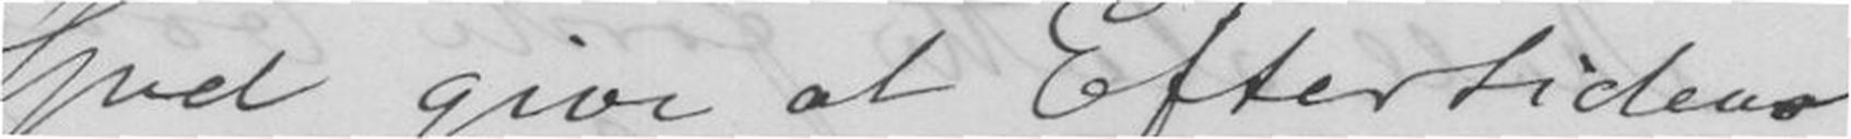Gud give at Eftertidene
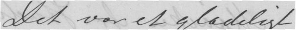Det var et glædeligt
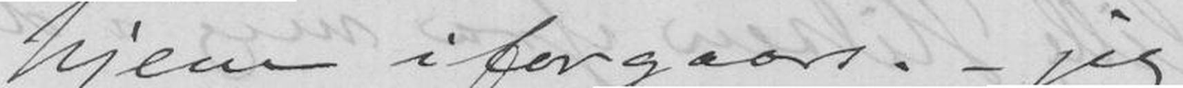hjem iforgaars; - jeg
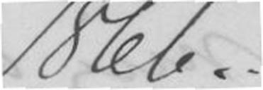1866.
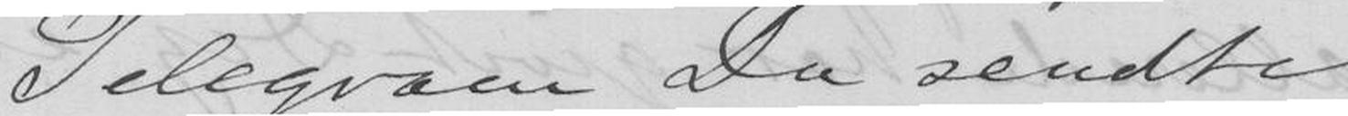Telegram Du sendte
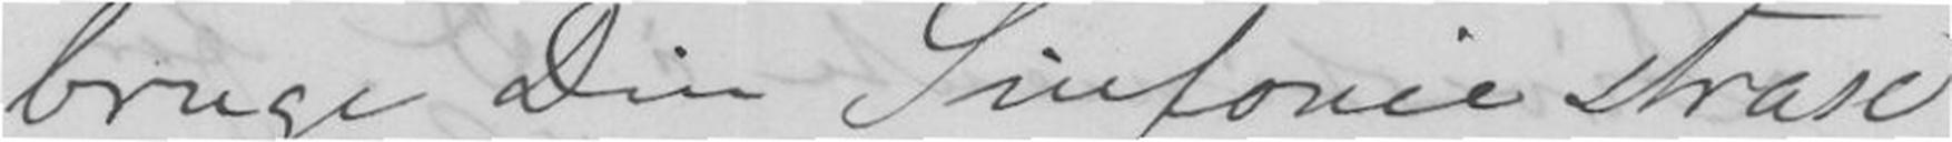bruge Din Sinfonie strax
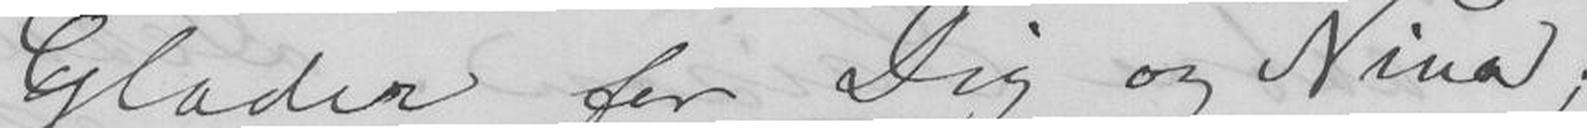Glæder for Dig og Nina;
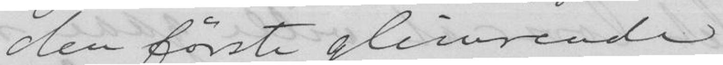den første glimrende
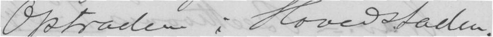Optræden i Hovedstaden.
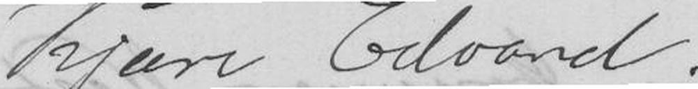Kjære Edvard.
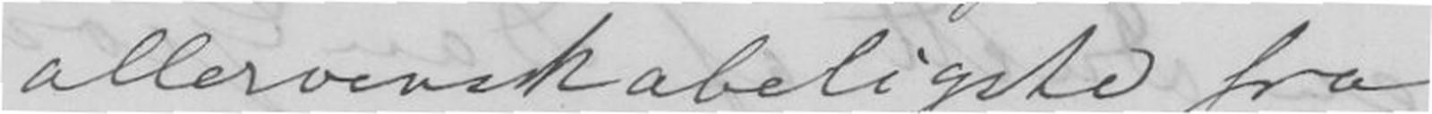allervenskabeligste fra
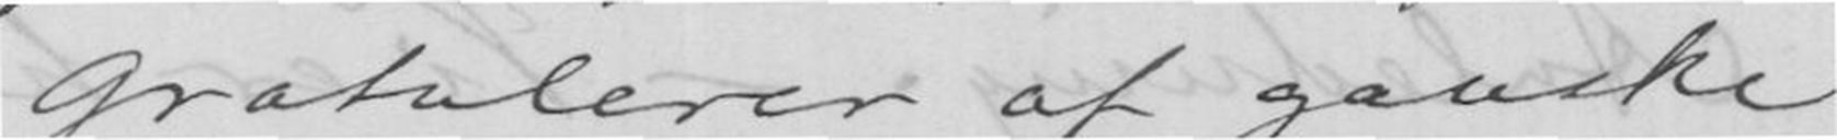gratulerer af ganske
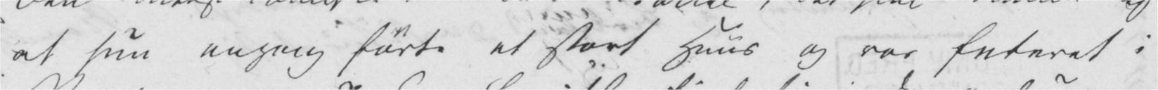at hun engang førte et stort Huus og var feteret i
